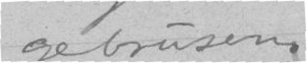gebrusen.
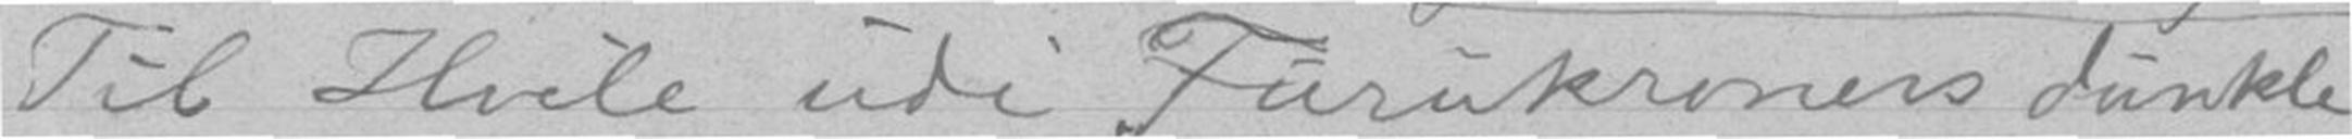Til Hvile udi Furukronens dunkle
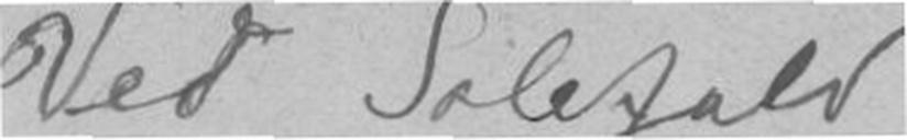Ved Solefald
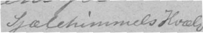Sjælehimmels Hvælv
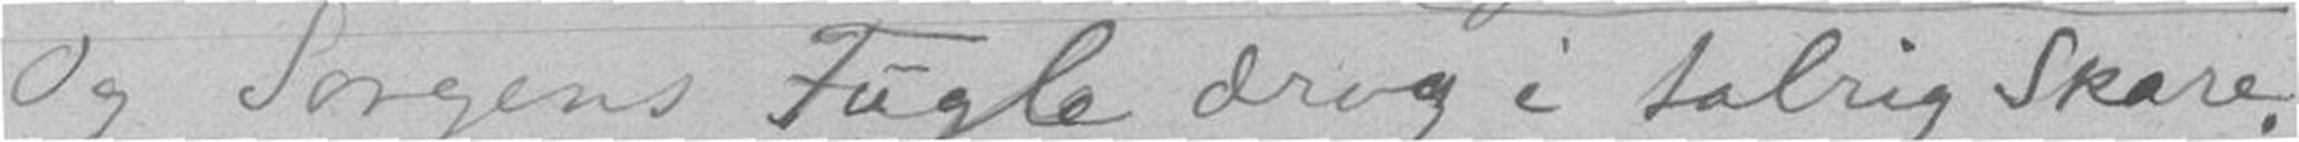Og Sorgens Fugle drog i talrig Skare.
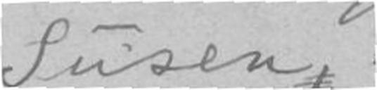Susen,.
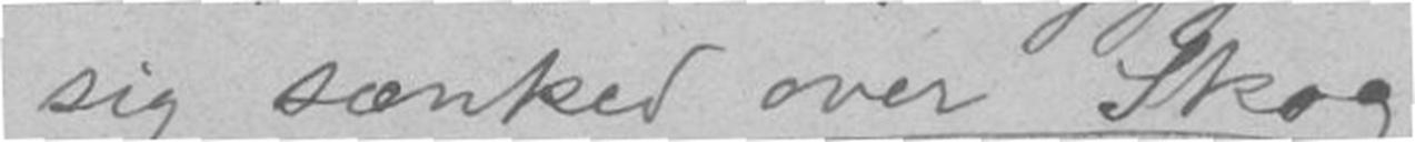sig sænker over Skog
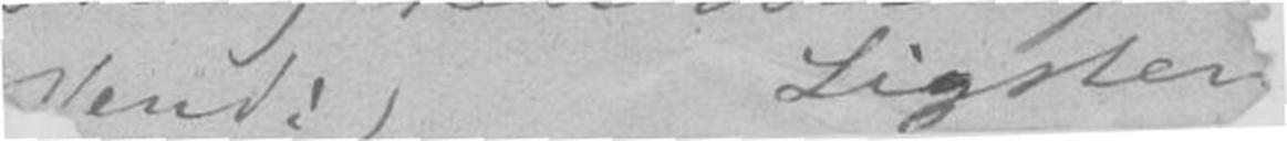( Vend!) Ligster
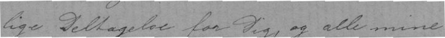lige Deltagelse for Dig, og alle mine
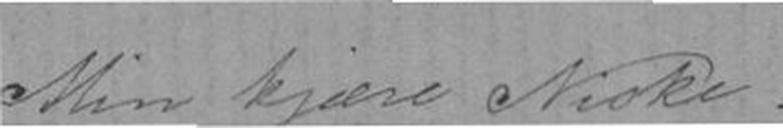Min kjære Niske!
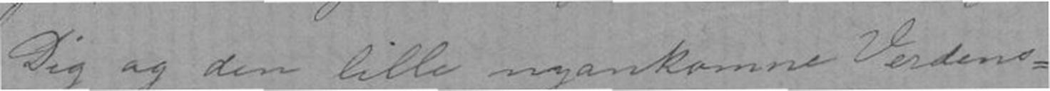Dig og det lille nyankomne Verdens-
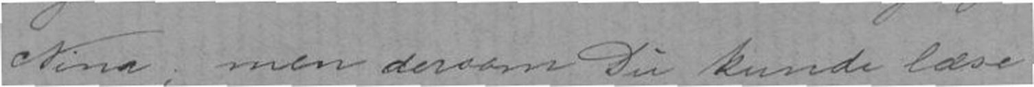Nina, men dersom Du kunde læse
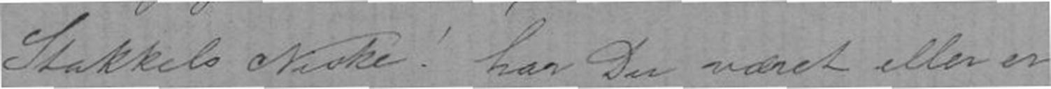Stakkels Niske! har Du været eller er
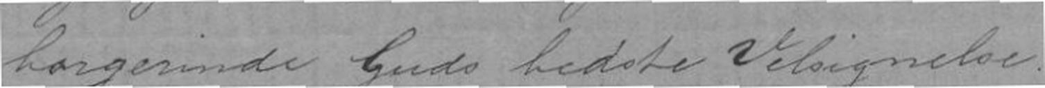borgerinde Guds bedste Velsignelse.
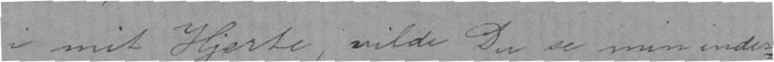i mit Hjerte, vilde Du se min inder-
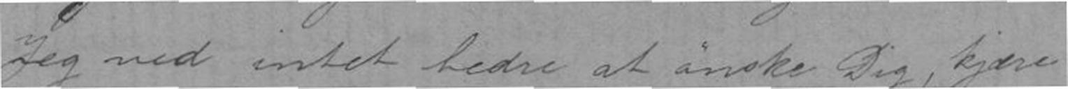Jeg ved intet bedre at ønske Dig, kjære
